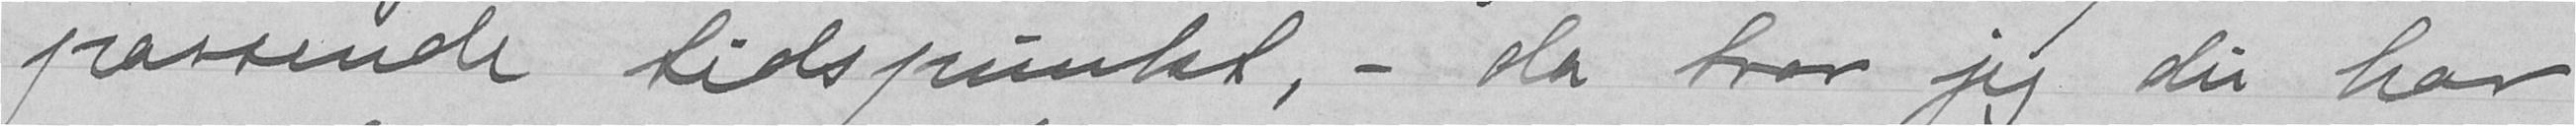passende tidspunkt, - da tror jeg du har
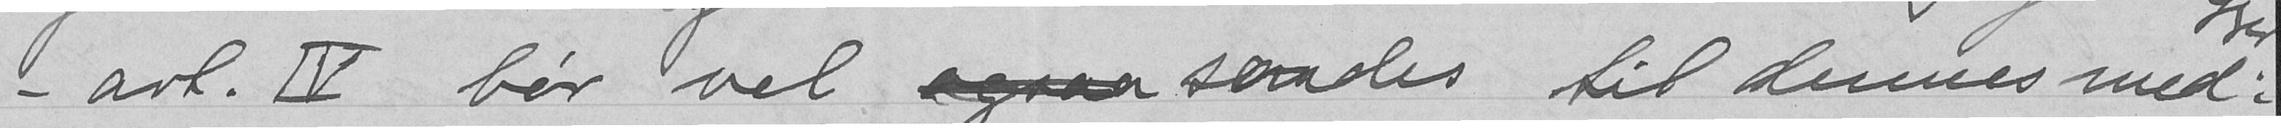- art. IV bør vel ogsaa sændes til dennes med-
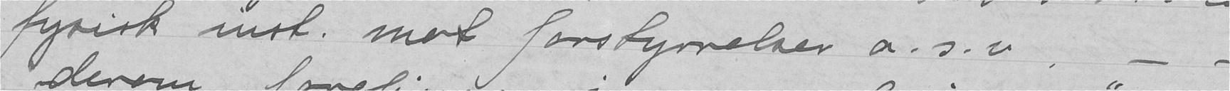fysisk inst. mod forstyrrelser o. s. v. -
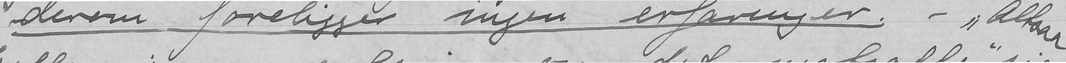derom foreligger ingen erfaringer. - Altsaa
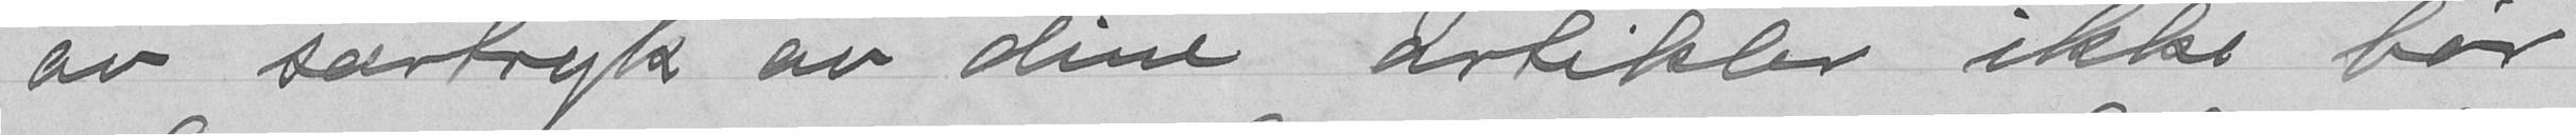av særtryk av dine artikler ikke bør
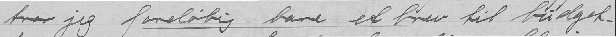tror jeg foreløbig bare et brev til budget-
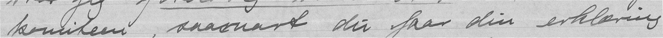komiteen, saasnart du faar din erklæring
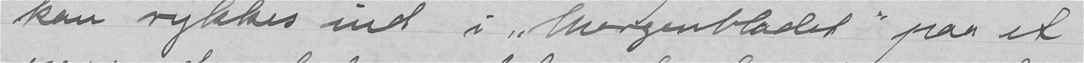kan rykkes ind i Morgenbladet paa et
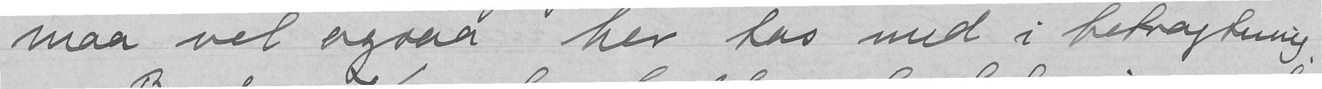maa vel ogsaa her tas med i betragtning.
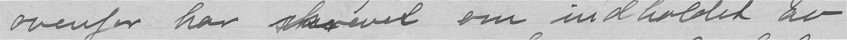ovenfor har skrevet om indholdet av
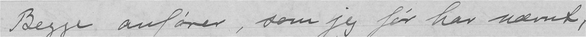Begge anfører, som jeg før har nævnt,
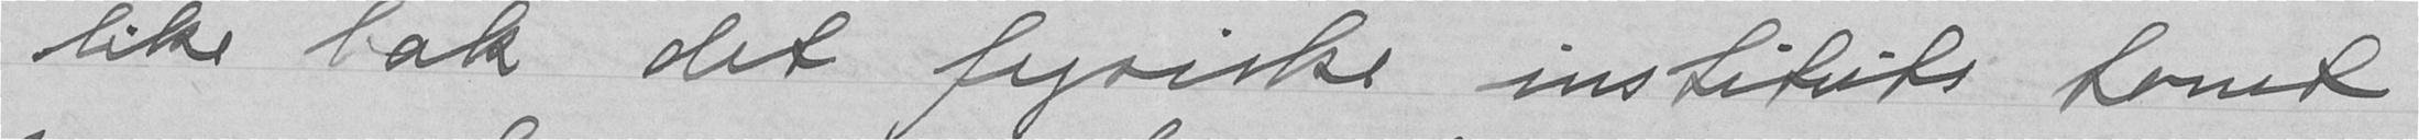like bak det fysiske instituts tomt
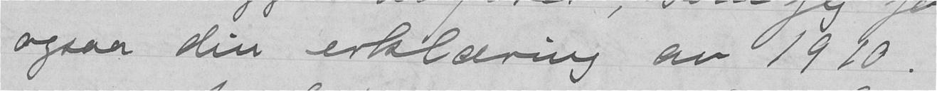ogsaa din erklæring av 1910.
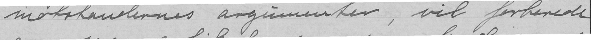motstandernes argumenter, vil forberede
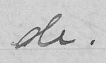de.
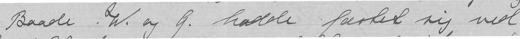Baade W. og G. hadde fæstet sig ved
